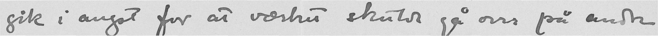gik i angst for at værket skulde gå over på andre
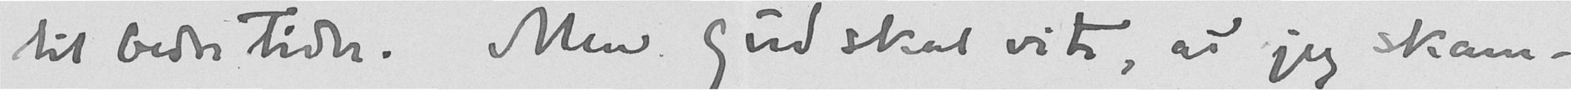til bedre tider. Men gud skal vite, at jeg skam-
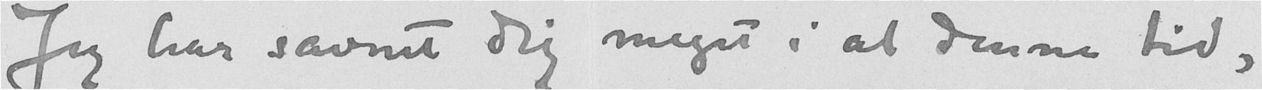Jeg har savnet dig meget i al denne tid,
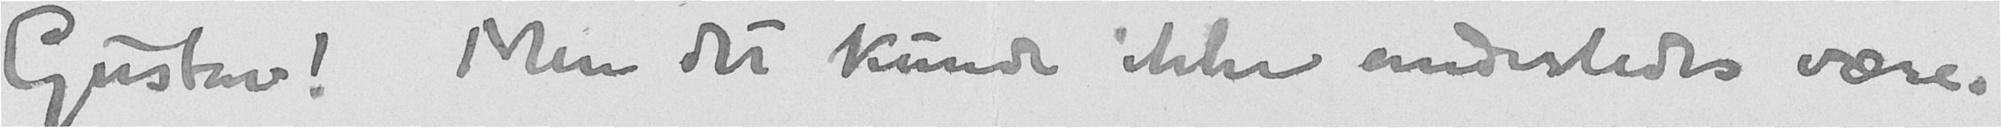Gustav! Men det kunde ikke anderledes være.
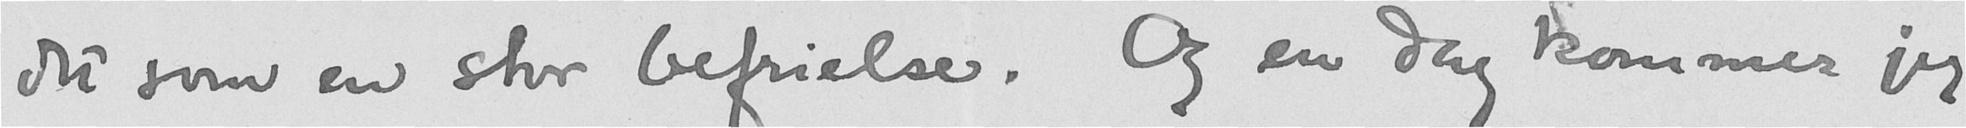det som en stor befrielse. Og en dag kommer jeg
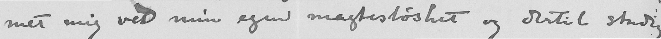met mig ved min egen magtesløshet og dertil stadig
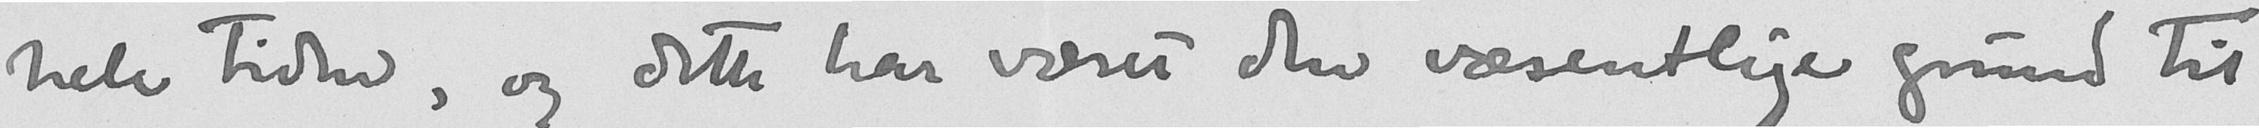hele tiden, og dette har været den væsentlige grund til
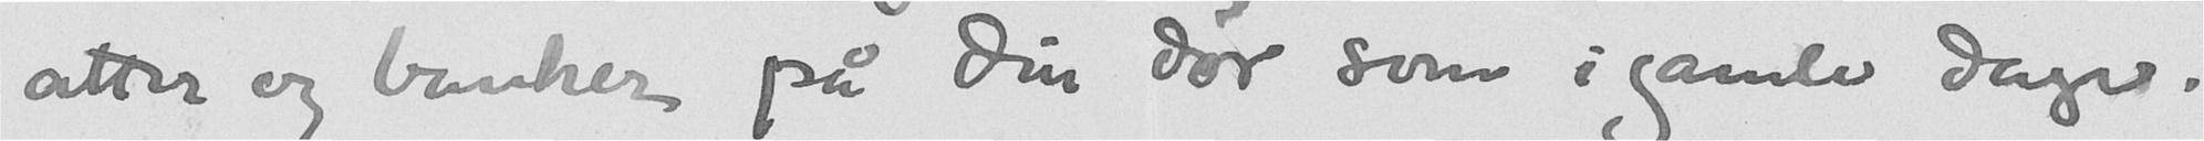atter og banker på din dør som i gamle dager.
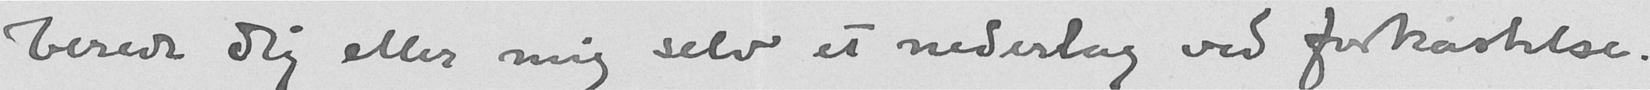berede dig eller mig selv et nederlag ved forkastelse.
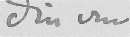Din ven
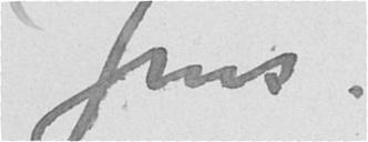Jens.
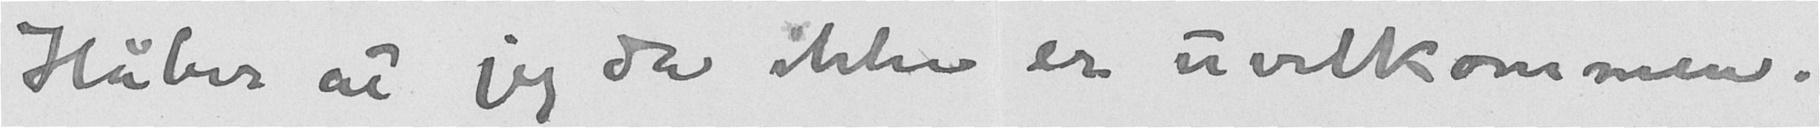Håber at jeg da ikke er uvelkommen.
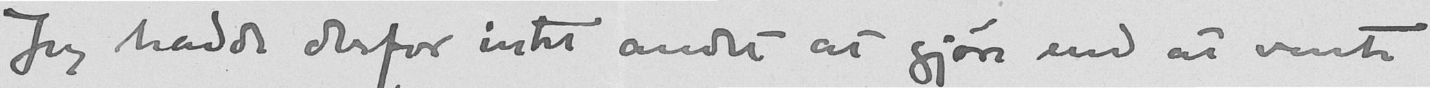Jeg hadde derfor intet andet at gjøre end at vente
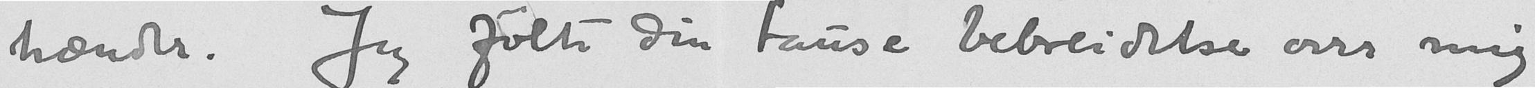hænder. Jeg følte din tause bebreidelse over mig
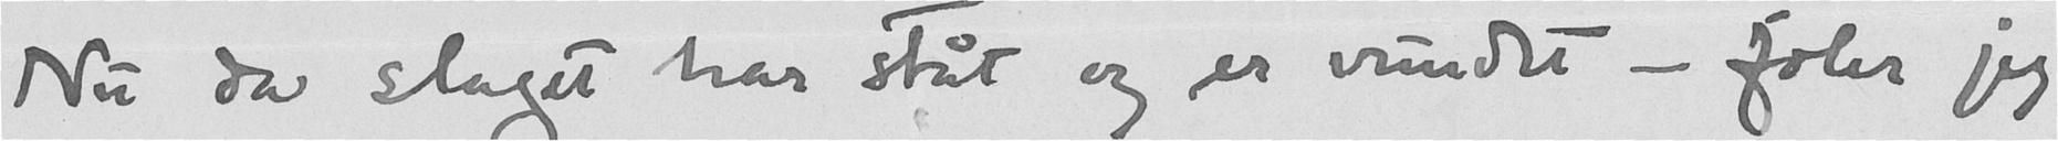Nu da slaget har ståt og er vundet - føler jeg
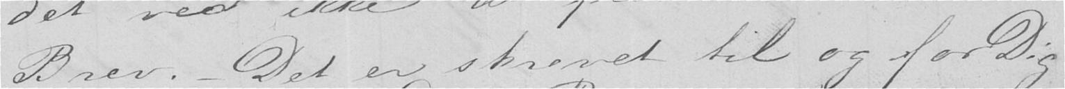Brev. - Det er skrevet til og for Dig
